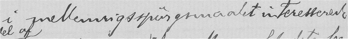i mellemrigsspørgsmaalet interesserede
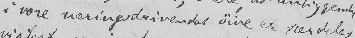i vore næringsdrivendes øine er særdeles
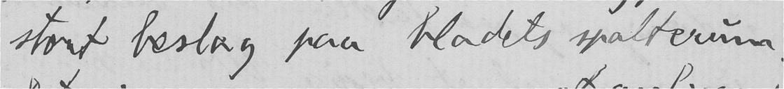stort beslag paa bladets spalterum.
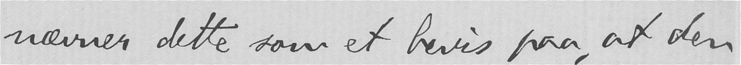nævner dette som et bevis paa, at den
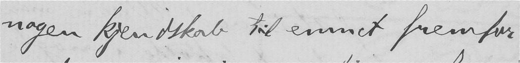nogen kjendskab til emnet fremfor
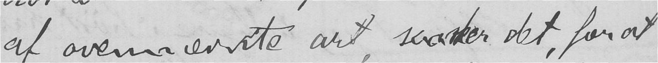af ovennævnte art, saa sker det, for at
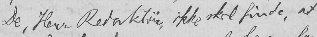De, Herr Redaktør, ikke skal finde, at
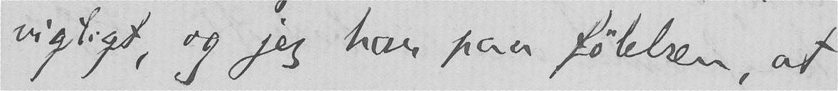vigtigt, og jeg har paa følelsen, at
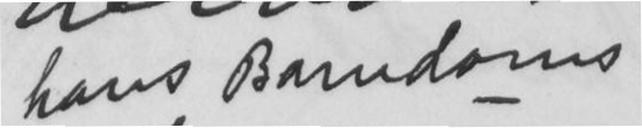hans Barndoms
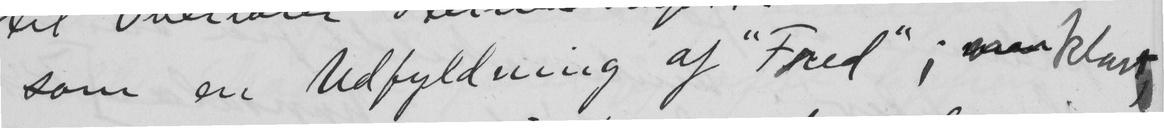som en Udfyldning af "Fred"; men klart
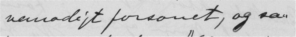vemodigt forsonet, og saa
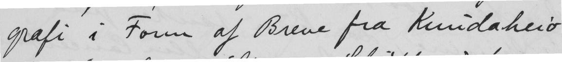grafi i Form af Breve fra Knudaheio
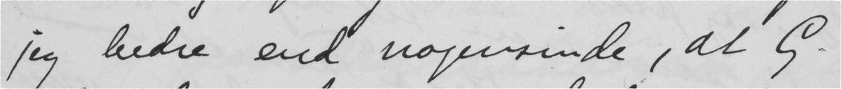jeg bedre end nogensinde, at G.
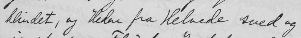blindet, og Heden fra Helvede sved og
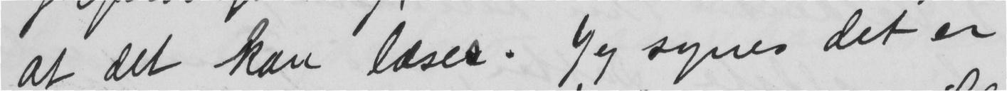at det kan læses. Jeg synes det er
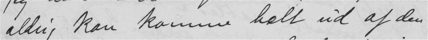aldrig kan komme helt ud af den
